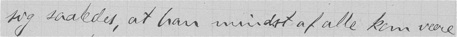sig saaledes, at han mindst af alle kan være
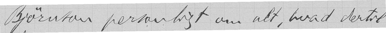Bjørnson personligt om alt, hvad dertil
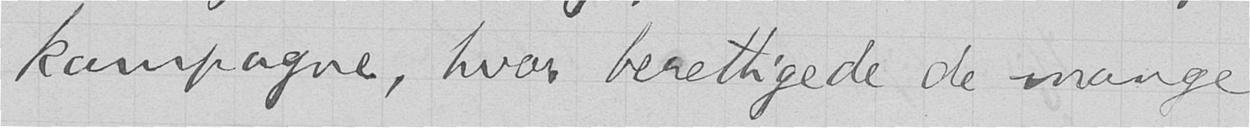kampagne, hvor berettigede de mange
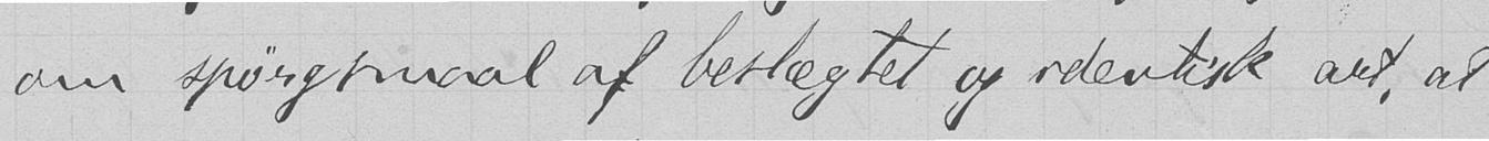om spørgsmaal af beslægtet og identisk art, at
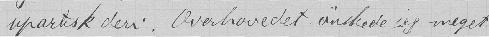upartisk deri. Overhovedet ønskede jeg meget
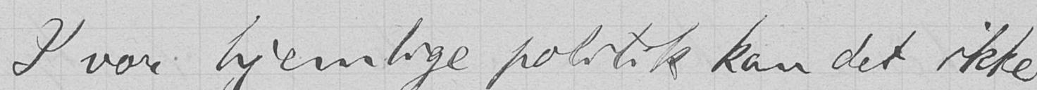I vor hjemlige politik kan det ikke
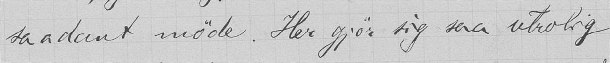saadant møde. Her gjør sig saa utrolig
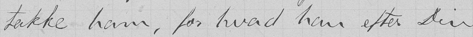takke ham, for hvad han efter Din
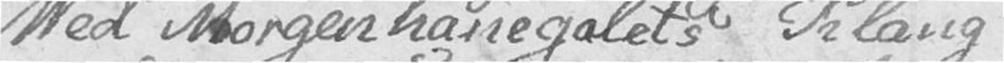Ved Morgenhanegalets Klang
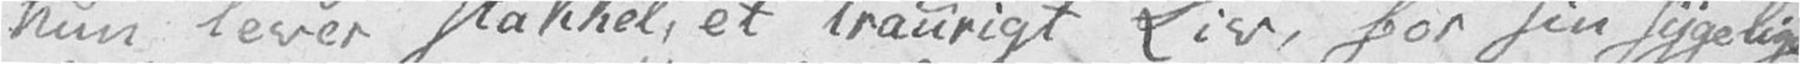hun lever stakkel, et traurigt Liv, for sin sygelige
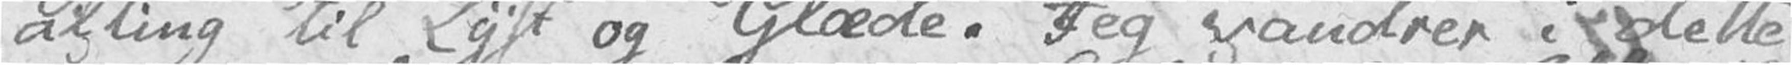alting til Lyst og Glæde. Jeg vandrer i dette
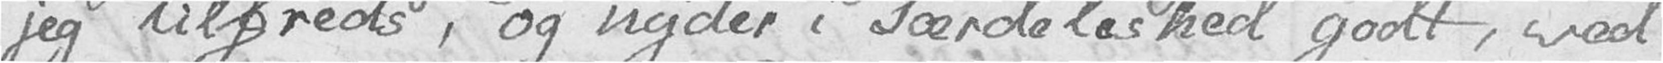jeg tilfreds, og nyder i Særdeleshed godt, ved
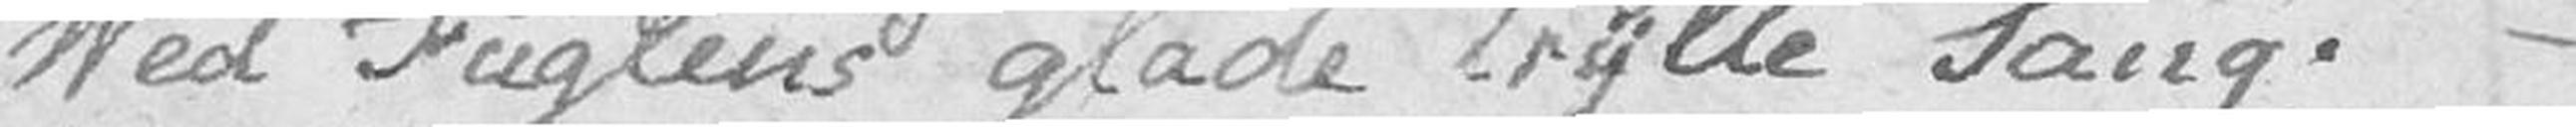Ved Fuglens glade trylle Sang. –
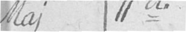Maj 11de
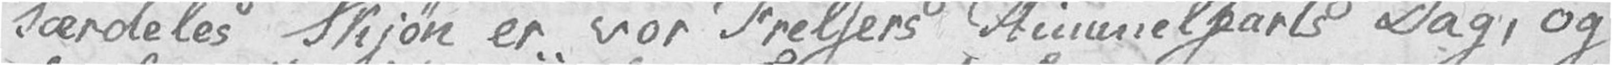Særdeles Skjøn er vor Frelsers Himmelfarts Dag, og
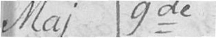Maj 9de
